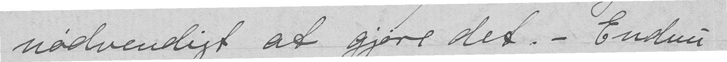nødvendigt at gjøre det. - Endnu
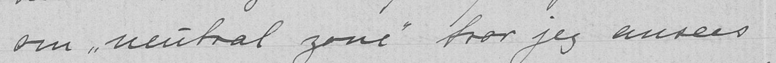om "neutral zone" tror jeg ansees
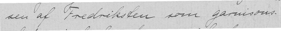sen af Fredriksten som garnisons-
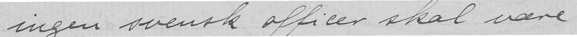ingen svensk officer skal være
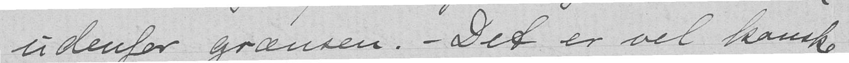udenfor grænsen. - Det er vel kanske
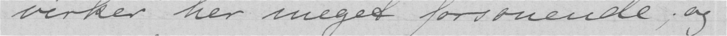virker her meget forsonende; og
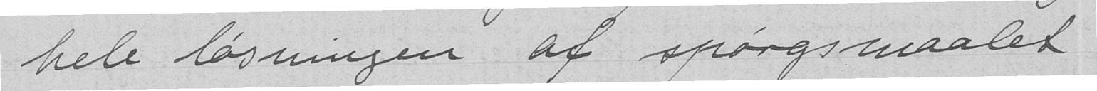hele løsningen af spørgsmaalet
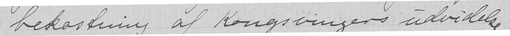bekostning af Kongsvingers udvidelse
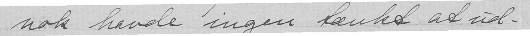nok havde ingen tænkt at ud-
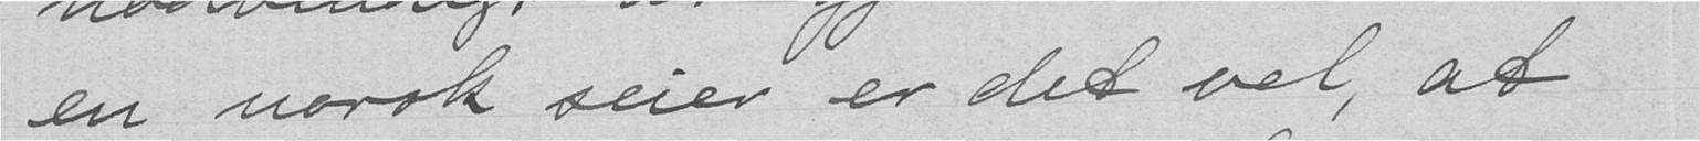en norsk seier er det vel, at
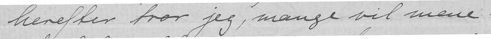herefter tror jeg, mange vil mene
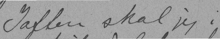Iaften skal jeg i
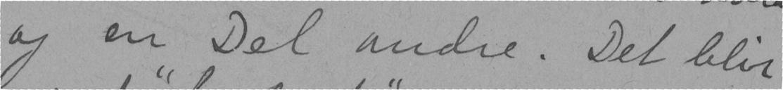og en Del andre. Det blir
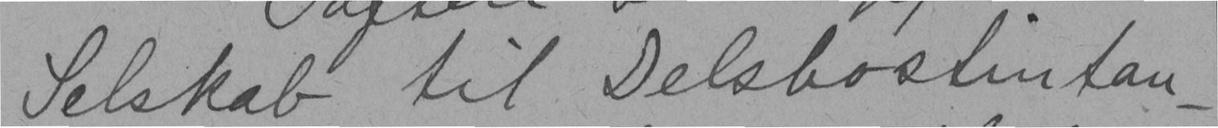Selskab til Delsbostintan -
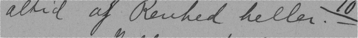altid af Renhed heller. —
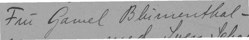Fru Gawel Blumenthal -
